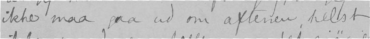ikke maa gaa ud om aftenen, helst
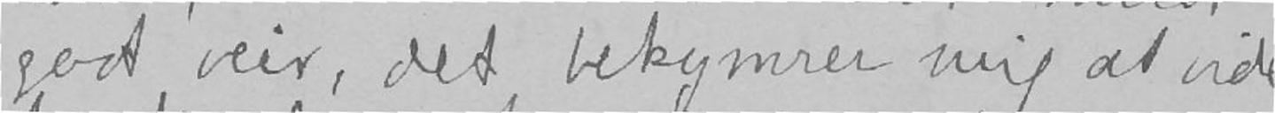godt veir, det bekymrer mig at vide
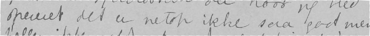opereret det er netop ikke saa godt, men
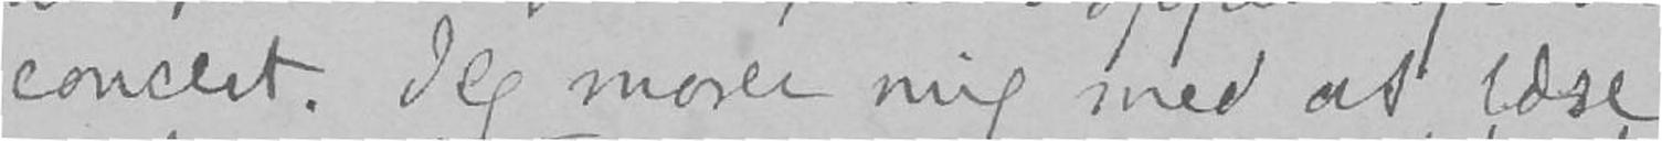concert. Jeg morer mig med at læse
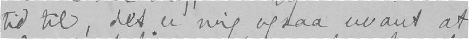tid til, det er mig ogsaa uvant at
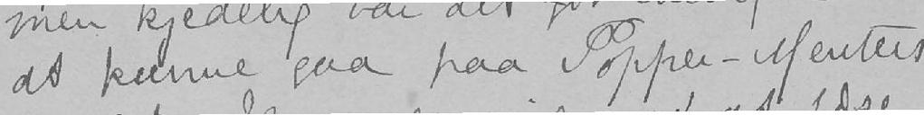at kunne gaa paa Popper-Menters
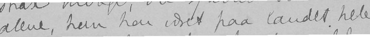alene, hun har været paa landet hele
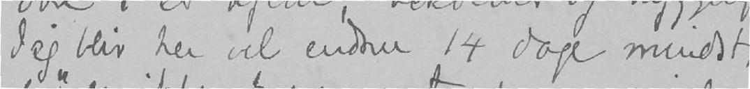Jeg blir her vel endnu 14 dage mindst,
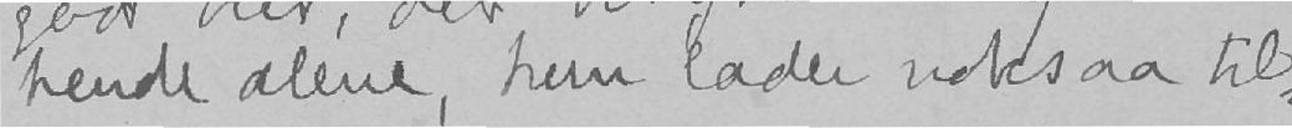hende alene, hun lader noksaa til-
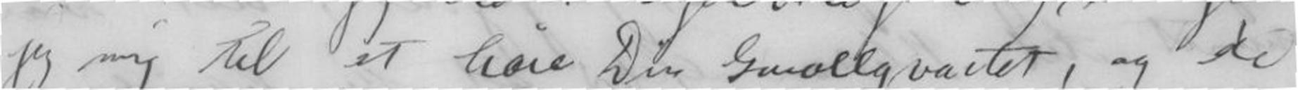jeg mig til at høre Din Gmollqvartet, og de
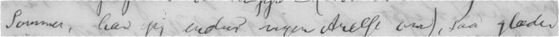Sommer, har jeg endnu ingen Anelse om), saa glæder
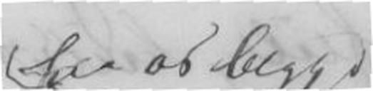fra os begge
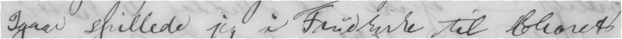Igaar spillede jeg i Fruekirke til Chorets
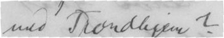med Tronhjem?
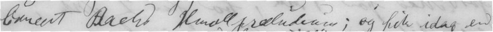concert Bachs Hmollpræludium; og fik idag en
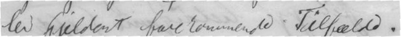ler skjeldent forekommende Tilfælde.
